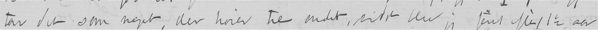tar det som noget, der hører til ondet, sidst blev jeg først efter 1 1/2 aar
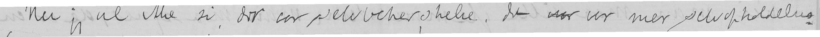Nei jeg vil ikke si, det var selvbeherskelse, det var var mer selvopholdelses-
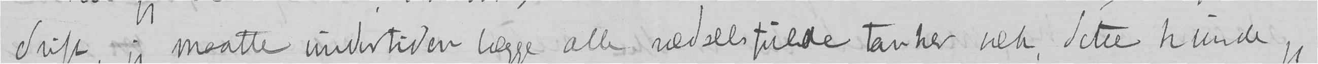drift, jeg maatte undertiden lægge alle rædselsfulde tanker væk, dette kunde jeg
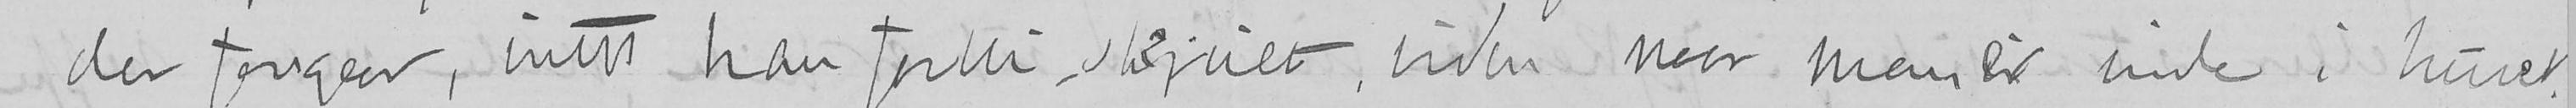der foregaar, intet kan forbli skjult, uden naar man er inde i huset.
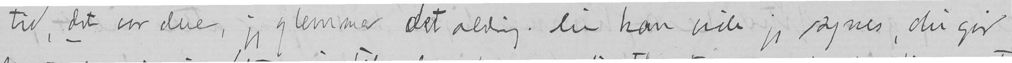tid, det var dere, jeg glemmer det aldrig. Du kan vide jeg synes, du gir
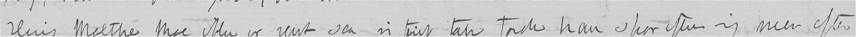Hvis Moltke Moe ikke er reist, saa si først tak fordi han spør efter mig men efter
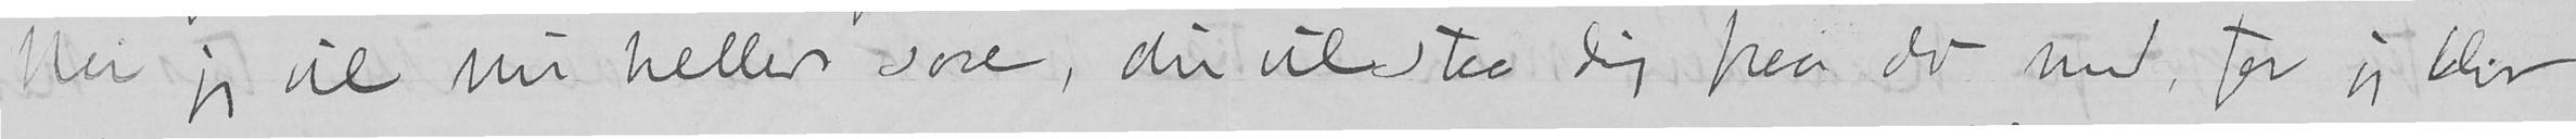Nei jeg vil nu heller sove, du vil staa dig paa det med, for jeg blir
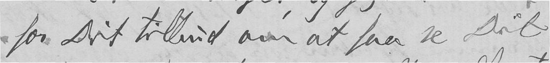for Dit tilbud om at faa se Dit
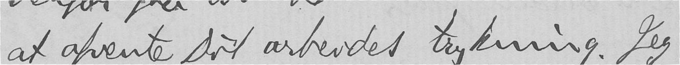at afvente Dit arbeides trykning. Jeg
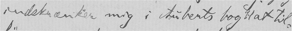indskrænker mig i Auberts bog til at til-
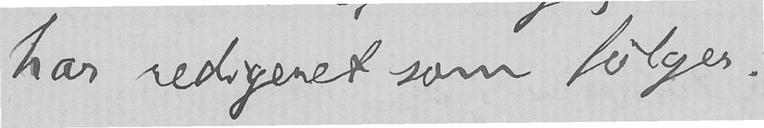har redigeret som følger:
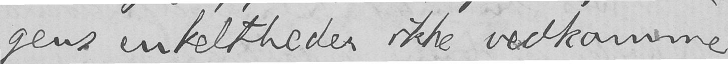gens enkeltheder ikke vedkomme
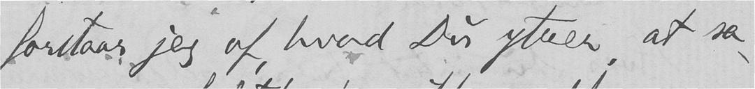forstaar jeg af, hvad Du yttrer, at sa-
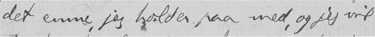det emne, jeg holder paa med, og jeg vil
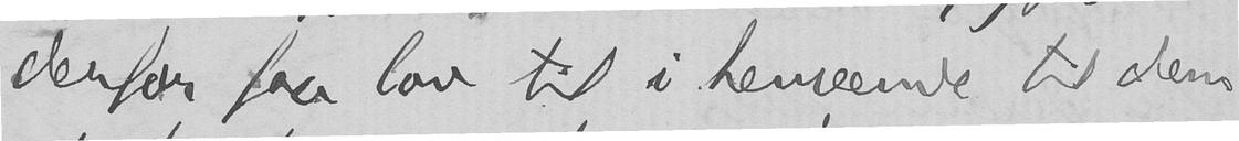derfor faa lov til i henseende til dem
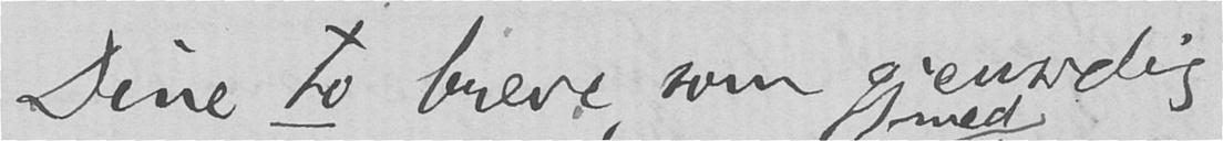Dine to breve, som gjensidig
